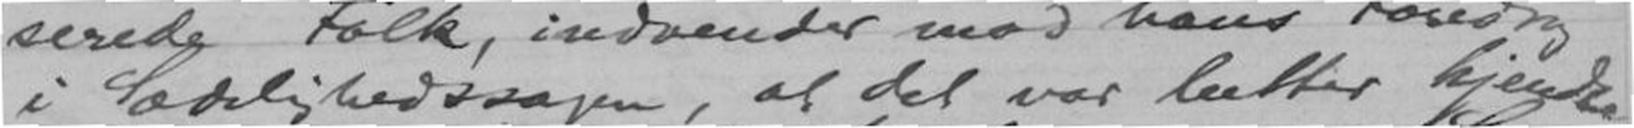i Sædelighedssagen, at det var lutter kjendte
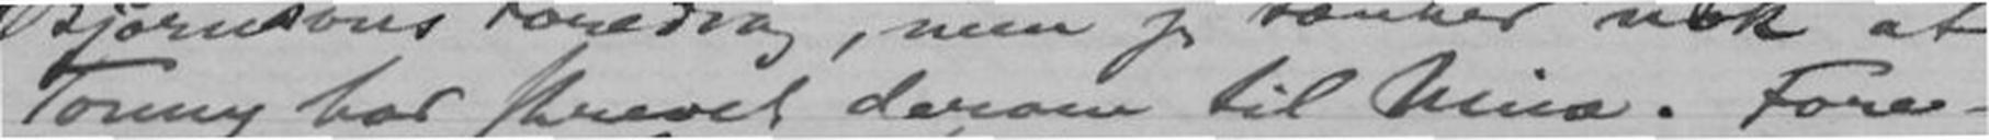Tonny har skrevet derom til Nina. Fore-
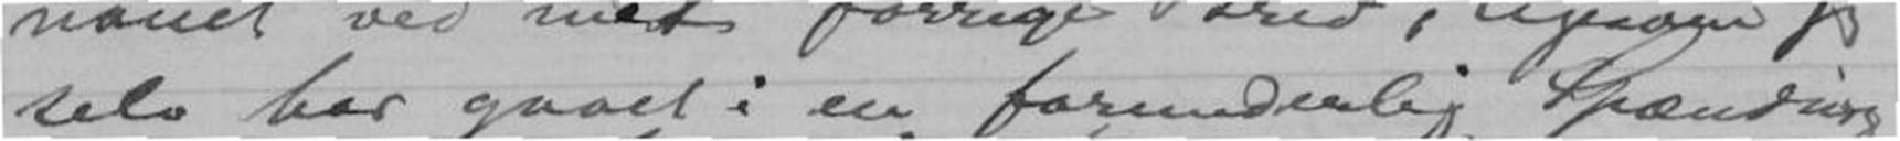selv har gaaet i en forunderlig Spænding
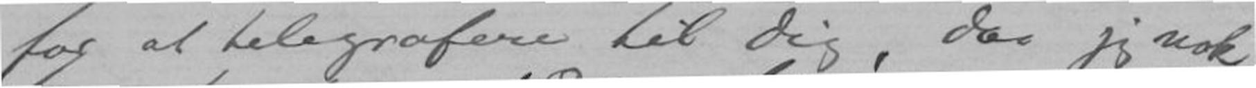for at telegrafere til Dig, da jeg nok
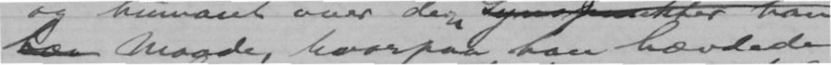hæv maade, hvorpaa han hævdede
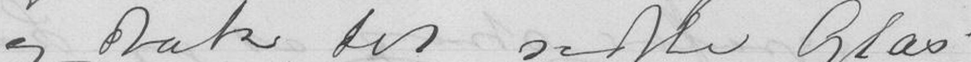og Drak det sidste Glas
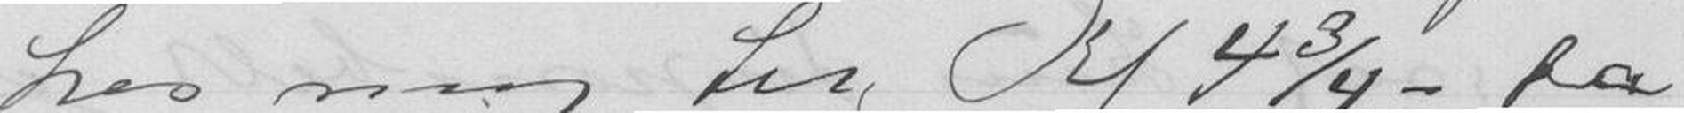hos mig til Kl 4¾ - Da
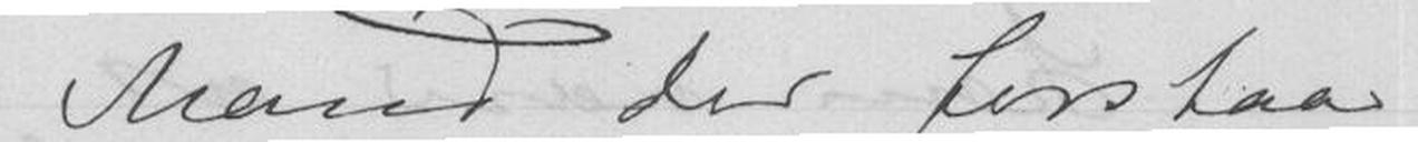Mand der forstaa
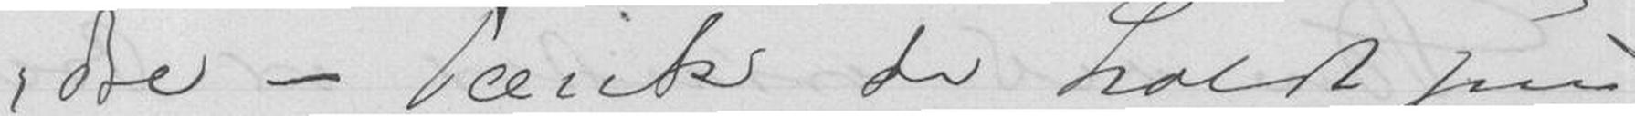dre - Tænk de holdt paa
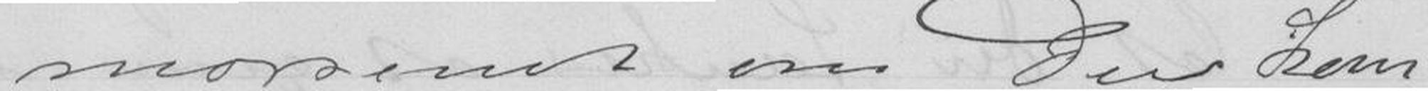morsomt om Du kom
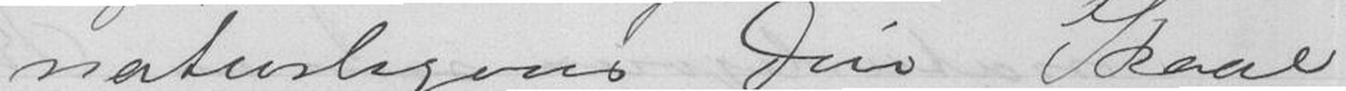naturligens Din Skaal
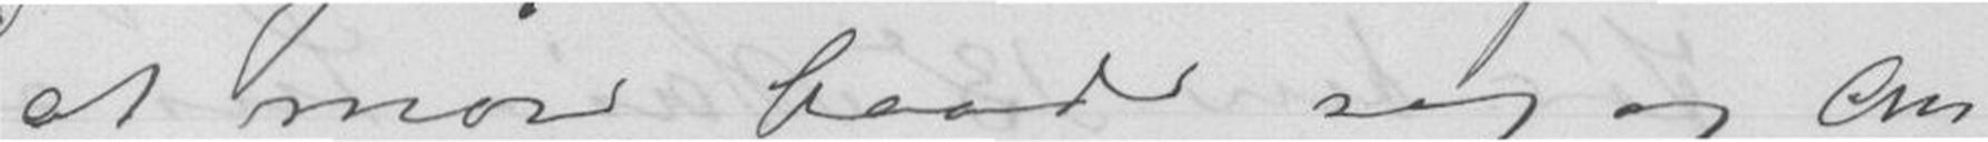at more baade sig og An-
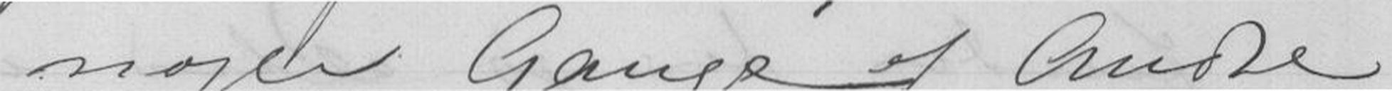nogle Gange af Andre
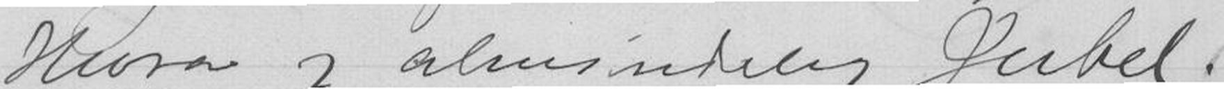Hura og almindelig Jubel.
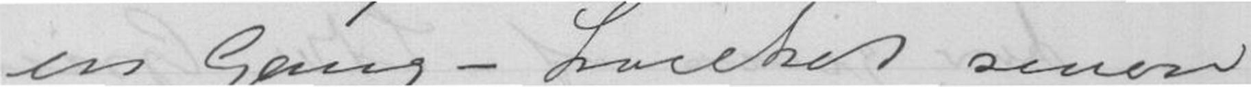en Gang - hvilket senere
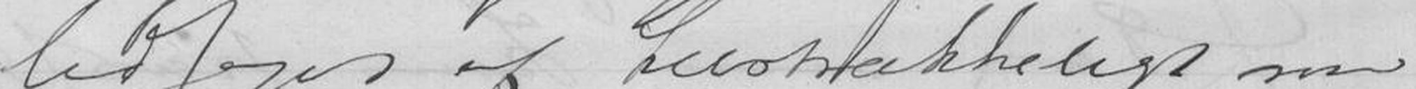ledsaget af tilstrækkeligt med
